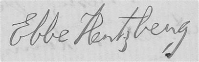Ebbe Hertzberg
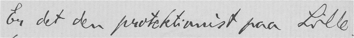Er det den protektionist paa Lille-
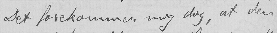Det forekommer mig dog, at den
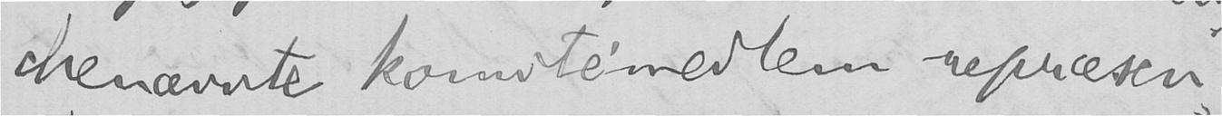che nævnte komitémedlem repræsen-
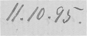11. 10. 95.
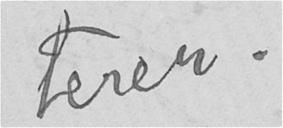terer.
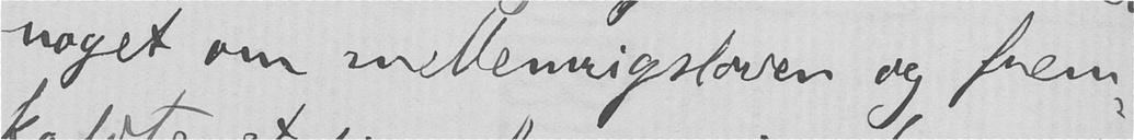noget om mellemrigsloven og frem-
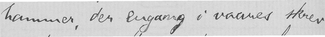hammer, der engang i vaares skrev
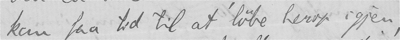kan faa tid til at løbe herop igjen,
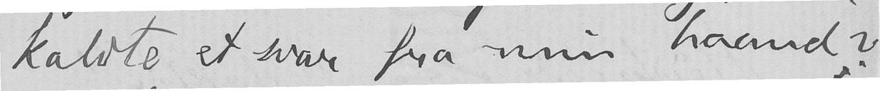kaldte et svar fra min haand?
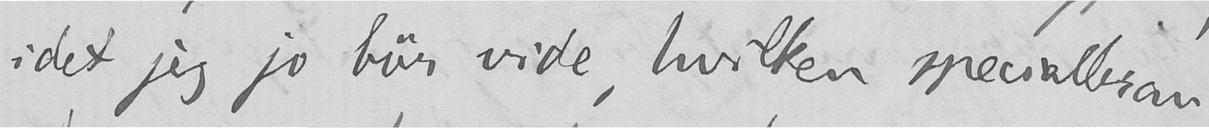idet jeg jo bør vide, hvilken specialbran-
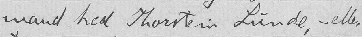mand hed Thorstein Lunde, - eller
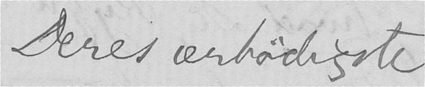Deres ærbødigste
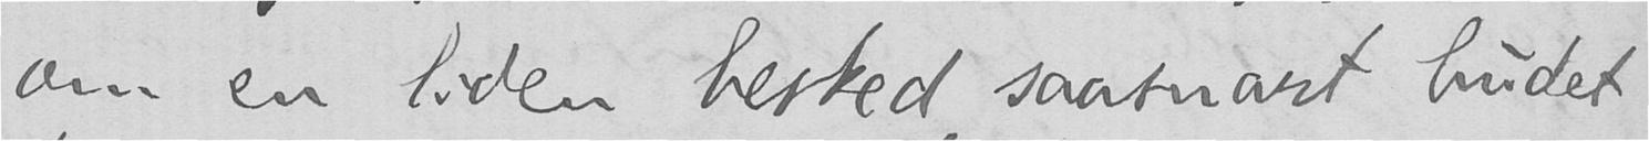om en liden besked, saasnart budet
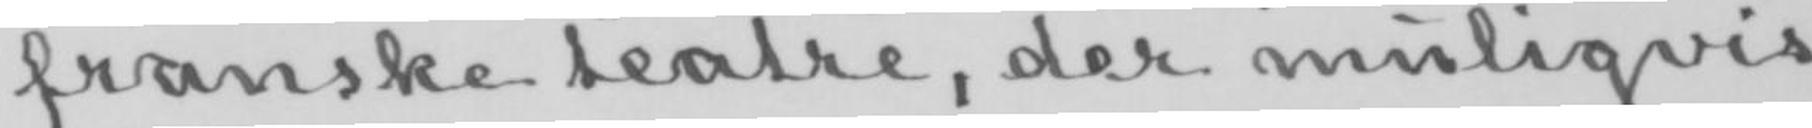franske teatre, der muligvis
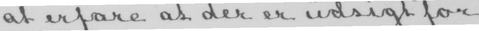at erfare at der er udsigt for
